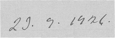23.9.1926.
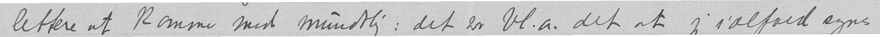lettere at komme med mundtlig: det er bl.a. det at jeg ialfald synes
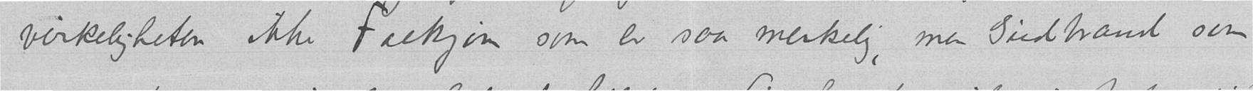virkeligheten ikke Falkjom som er saa merkelig, men Gudbrand som
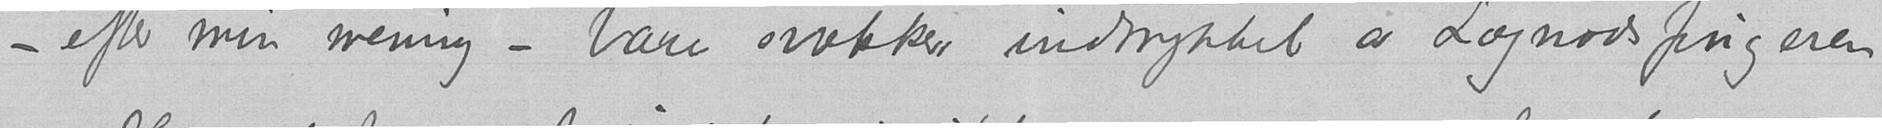– efter min mening – bare svækker indtrykket av Lagnadsfingeren
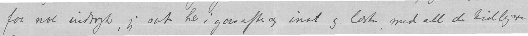faa noe indtryk, jeg sat her i gaaraftes og inat og læste, med alle de tidligere
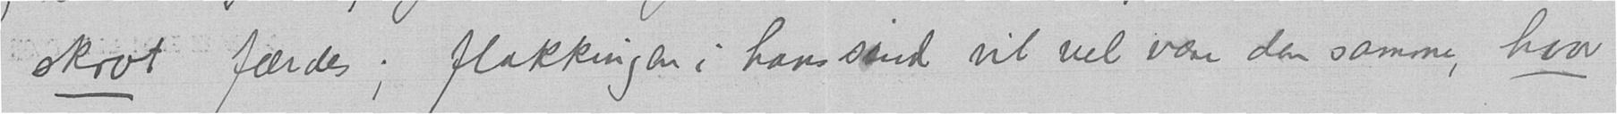skrot færdes; flakkingen i hans sind vil vel være den samme, hvor
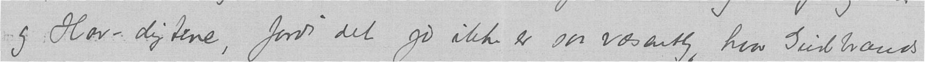og Hav-digtene, fordi det jo ikke er saa væsentlig, hvor Gudbrands
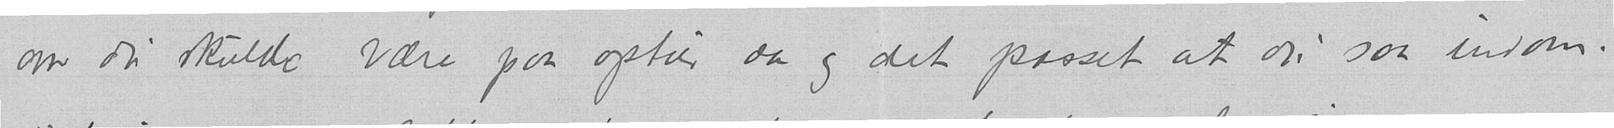om du skulde være paa optur da og det passet at du saa indom.
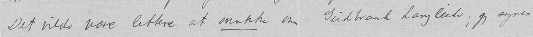Det vilde være lettere at snakke om Gudbrand Langleite; jeg synes
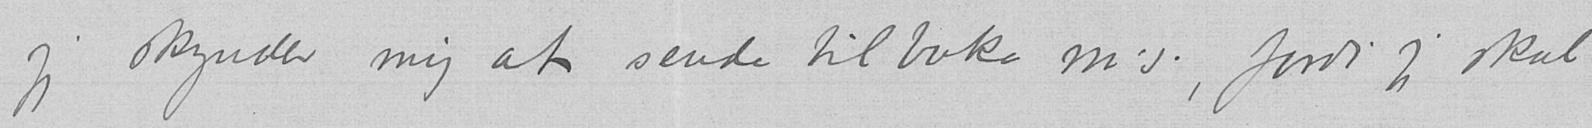jeg skynder mig at sende tilbake m.s., fordi jeg skal
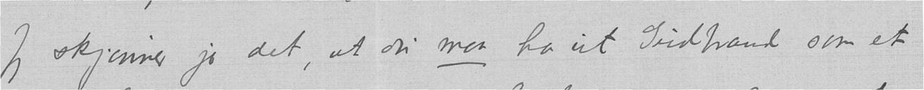Jeg skjønner jo det, at du maa ha ut Gudbrand som et
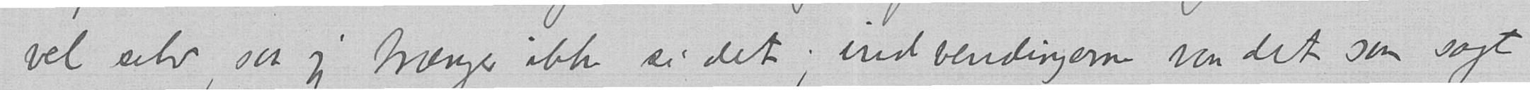vel selv, saa jeg trænger ikke si det; indvendingerne var det som sagt
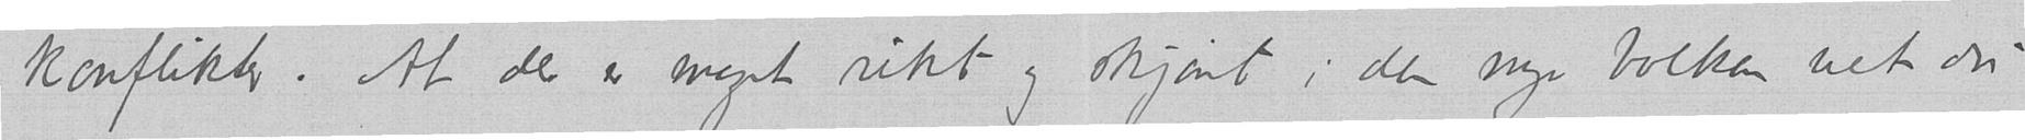konflikter. At der er meget rikt og skjønt i den nye bolken vet du
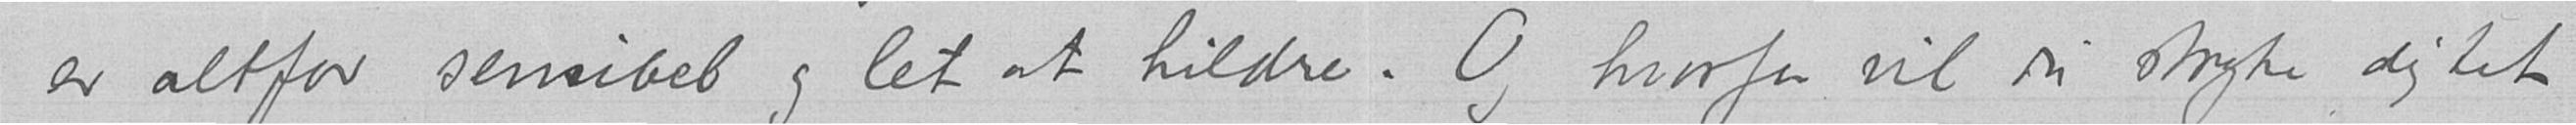er altfor sensibel og let at hildre. Og hvorfor vil du stryke digtet
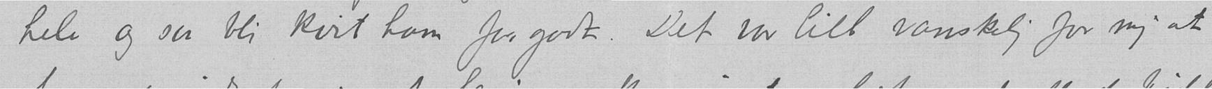hele og saa bli kvit ham for godt. Det var litt vanskelig for mig at
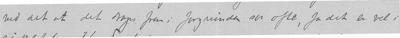ved det at det drages frem i forgrunden saa ofte, for det er vel i
